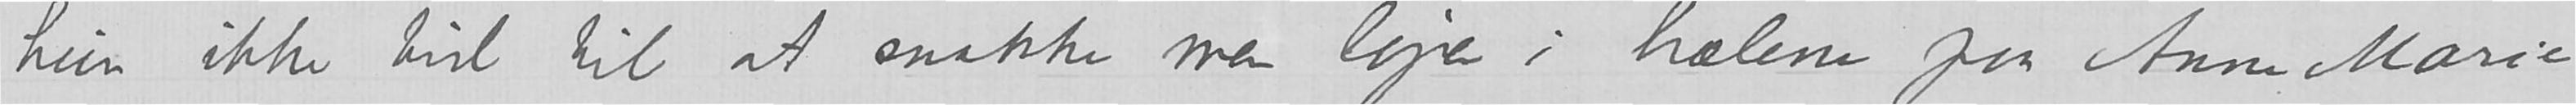hun ikke tid til at snakke men løper i hælene paa Anne Marie
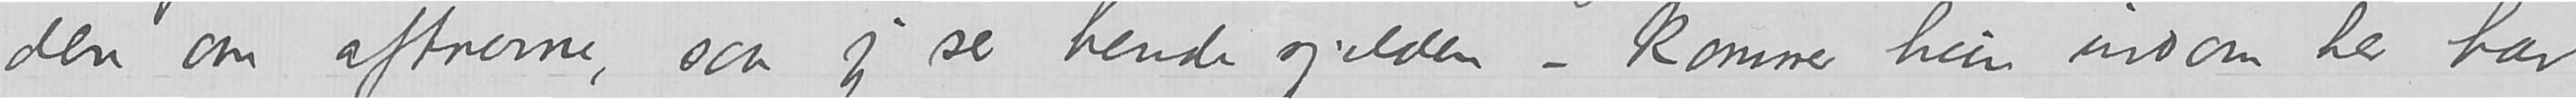den om aftnerne, saa jeg ser hende sjelden – kommer hun indom her har
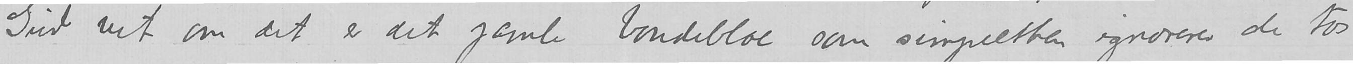Gud vet om det er det gamle bondebloe som simpelthen ignorerer de tos
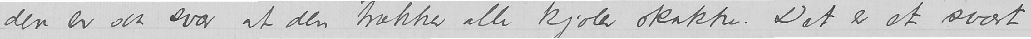den er saa svær at den trækker alle kjoler skakke. Det er et svært
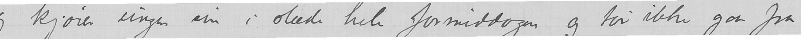og kjører ungen sin i slæde hele formiddagen og tør ikke gaa fra
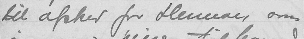til afsked for Herman, som
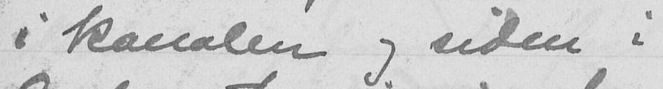i kanalen og siden i
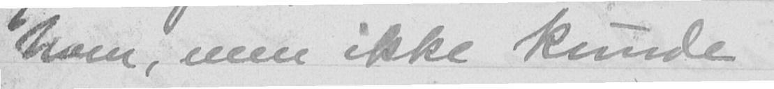ham, men ikke kunde.
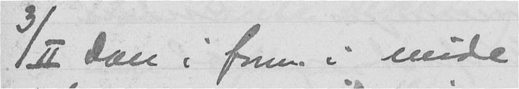3/II Don i form. i møde
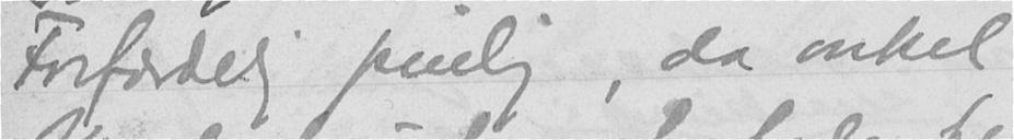Forfærdelig pinlig, da onkel
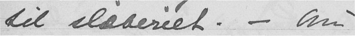til sliberiet. - Om
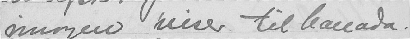imorgen reiser til Canada.
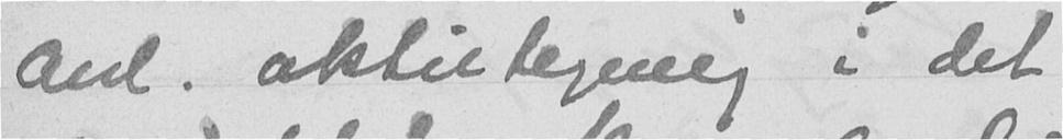anl. aktietegning i det
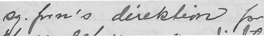sag. forn's direktion for
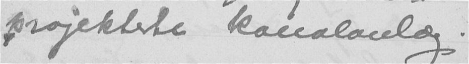projekterte kanalanlæg.
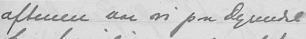aftenen var vi paa Dyrendal
